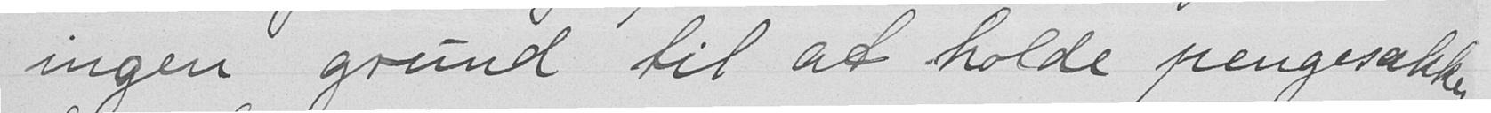ingen grund til at holde pengesækken
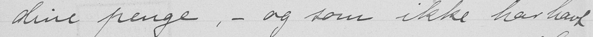dine penge, - og som ikke har havt
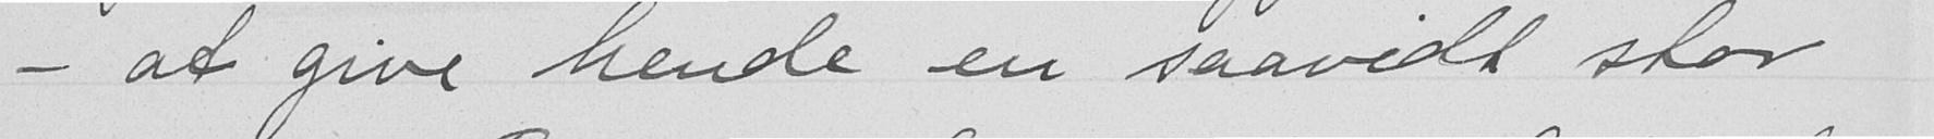- at give hende en saavidt stor
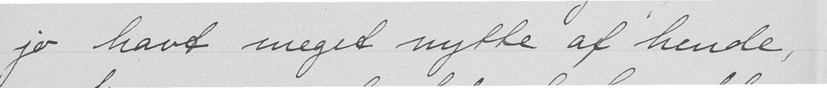jo havt meget nytte af hende,
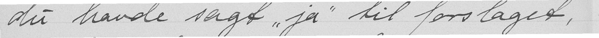du havde sagt "ja" til forslaget,
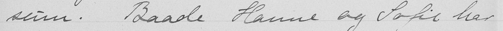sum. Baade Hanne og Sofie har
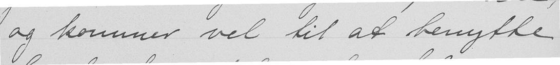og kommer vel til at benytte
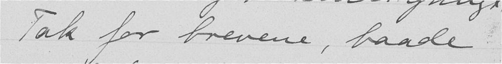Tak for brevene, baade
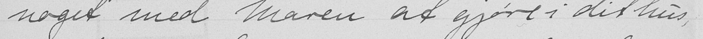noget med Maren at gjøre i dit hus,
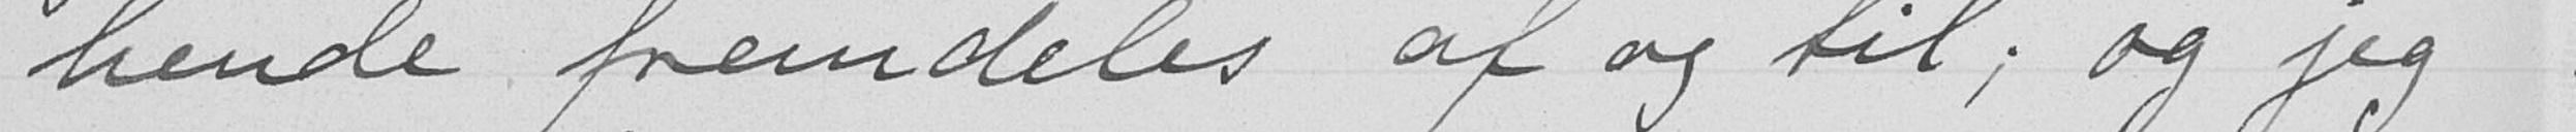hende fremdeles af og til; og jeg
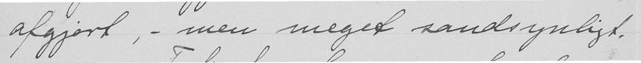afgjort, - men meget sandsynligt.
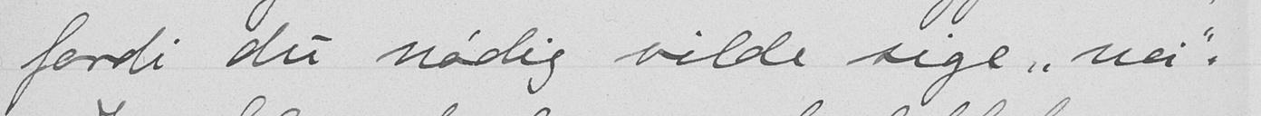fordi du nødig vilde sige "nei".
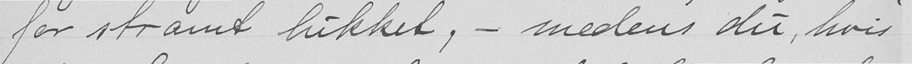for stramt lukket, - medens du, hvis
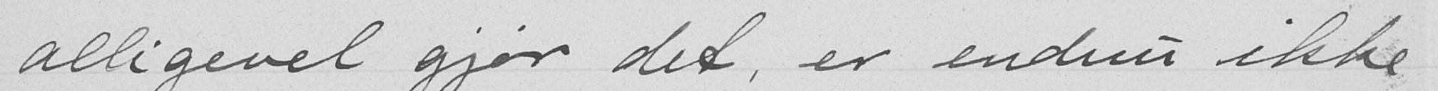alligevel gjør det, er endnu ikke
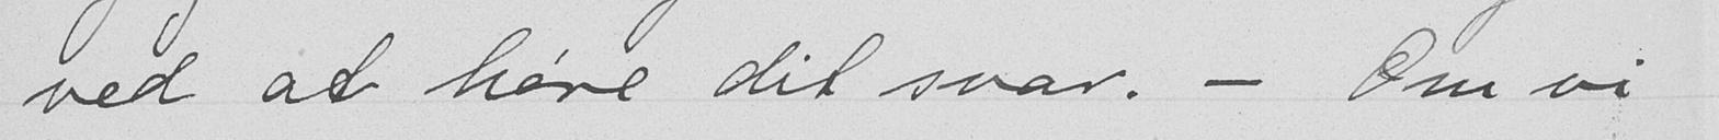ved at høre dit svar. - Om vi
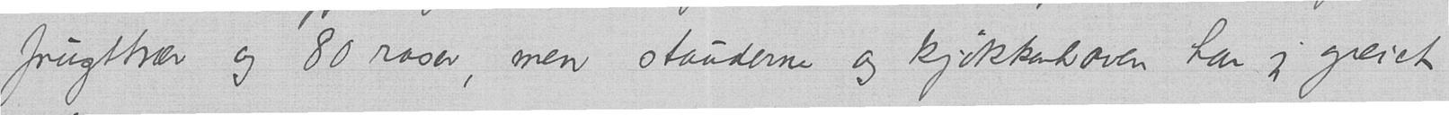frugttrær og 80 roser, men stauderne og kjøkkenhaven har jeg greiet
